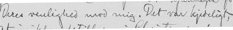Deres venlighed mod mig. Det var kjedeligt,
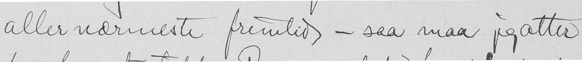aller nærmeste fremtid, - saa maa jeg atter
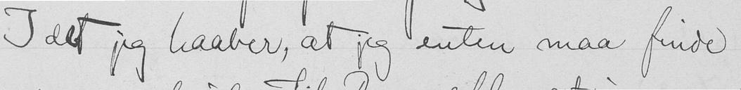I det jeg haaber, at jeg enten maa finde
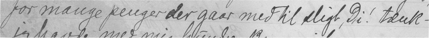for mange penger der gaar med til sligt, Di! tænk -
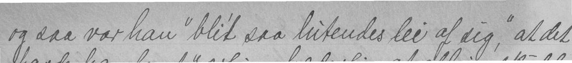og saa var han "bli't saa lutendes lei af sig, "at det
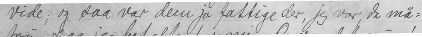vide; og saa var dem jo fattige der, jeg var da må-
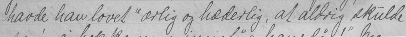havde han lovet "ærlig og hæderlig, at aldrig skulde
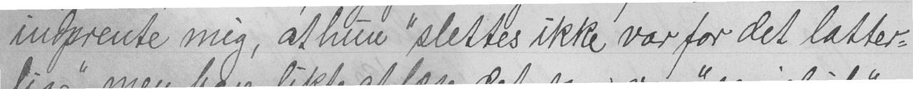indprente mig, at hun "slettes ikke var for det latter-
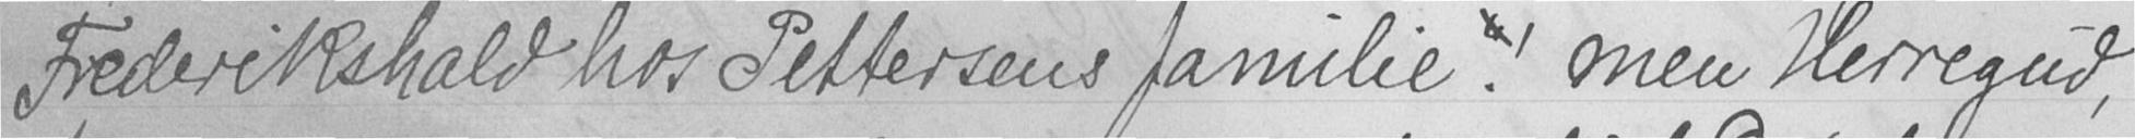Frederikshald hos Pettersens familie"! men Herregud,
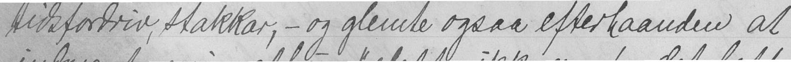tidsfordriv, stakkar, - og glemte ogsaa efterhaanden at
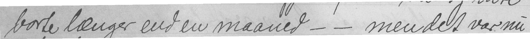borte længer end en maaned - - men det var nu
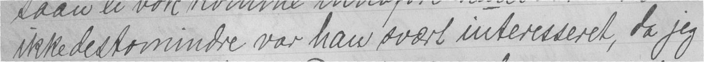ikke destomindre var han svært interesseret, da jeg
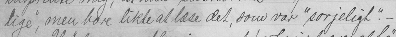lige", men bare likte at læse det, som var "sørgeligt." -
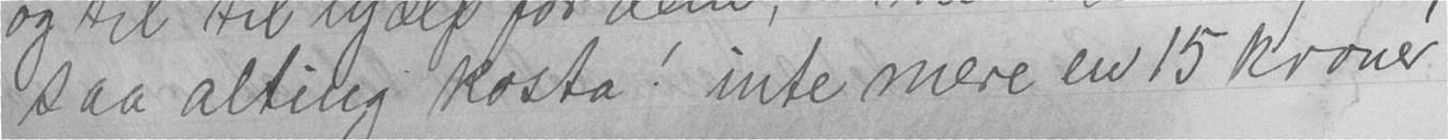saa altilng kosta! inte mere en 15 kroner
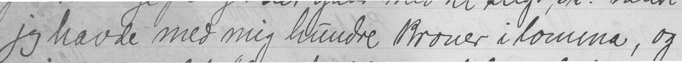jeg havde med mig hundre kroner i lomma, og
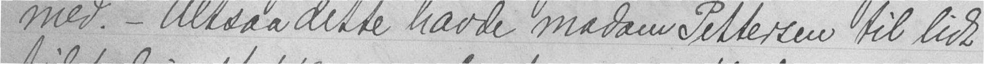med. - Altsaa dette havde madam Pettersen til lidt
